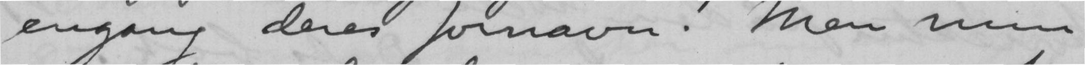engang deres fornavn. Men min
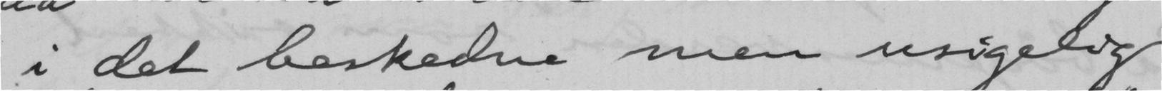i det beskedne men usigelig
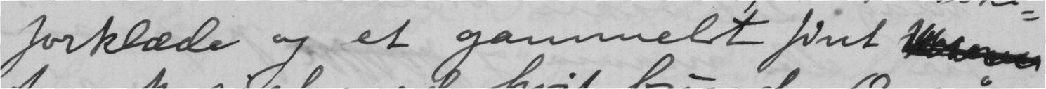forklæde og et gammelt fint
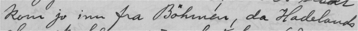kom jo inn fra Bøhmen, da Hadelands
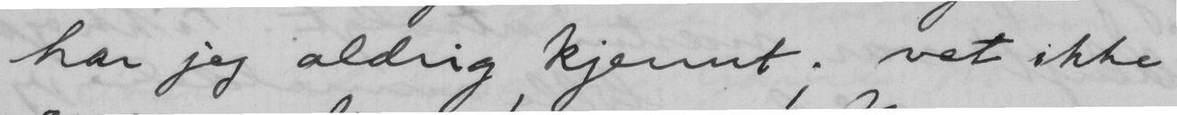har jeg aldrig kjennt; vet ikke
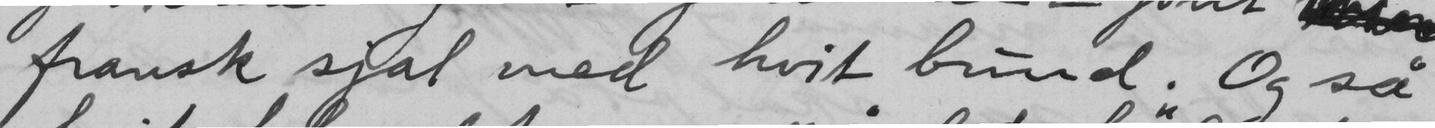fransk sjal med hvit bund. Og så
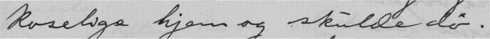koselige hjem og skulde dø.
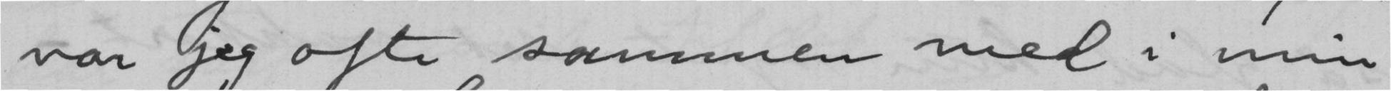var jeg ofte sammen med i min
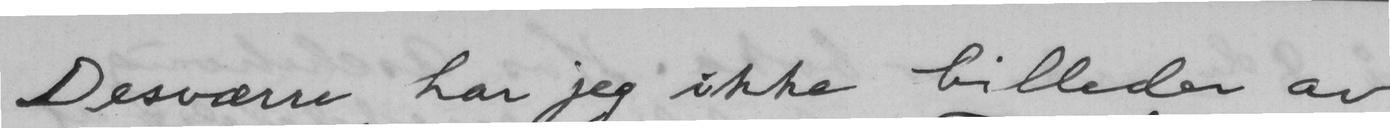Desværre har jeg ikke billeder av
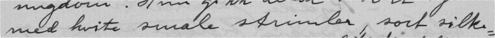med hvite smale strimler, sort silke-
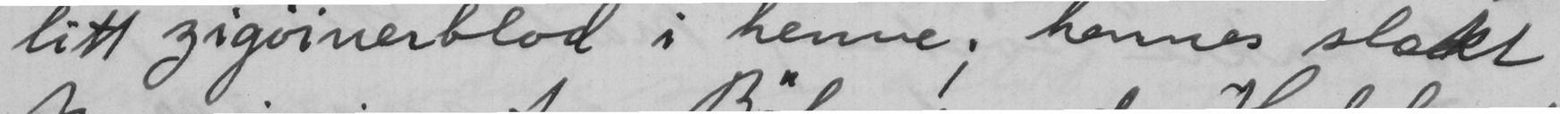litt sigøinerblod i henne; hennes slekt
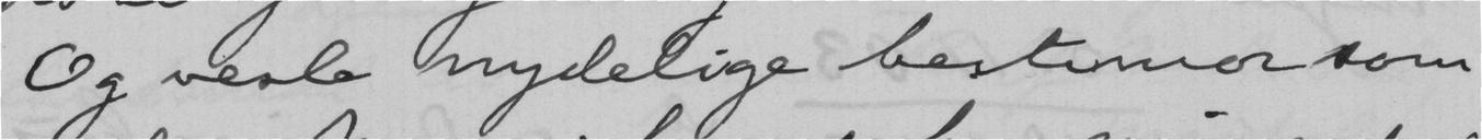Og vesle nydelige bestemer som
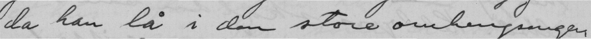da han lå i den store omhengsengen
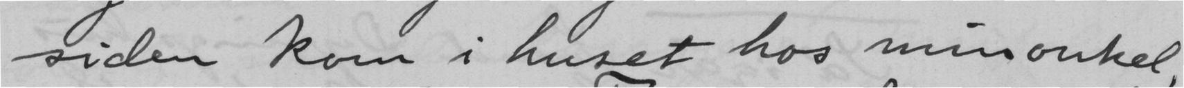siden kom i huset hos min onkel,
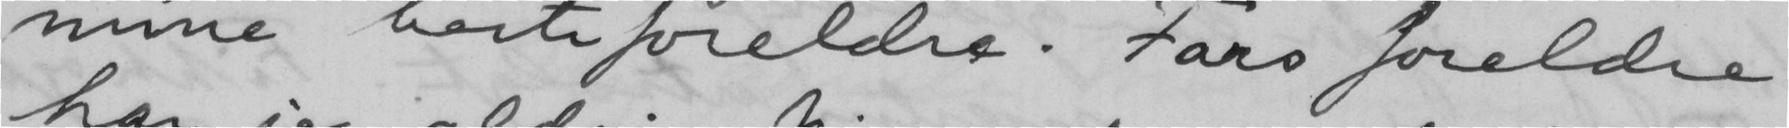mine besteforeldre. Fars foreldre
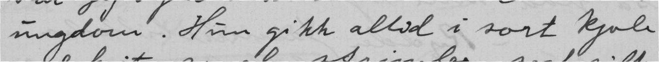ungdom. Hun gikk altid i sort kjole
